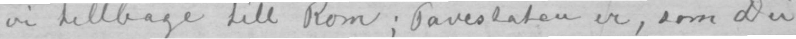vi tillbage till Rom; Pavestaten er, som Du
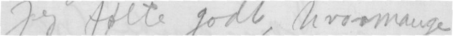Jeg følte godt, hvormange
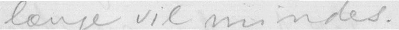længe vil mindes.
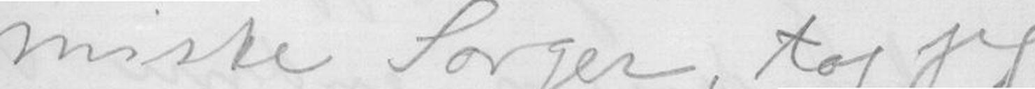miske Sorger, tog jeg
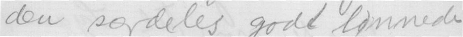den særdeles godt lønnede
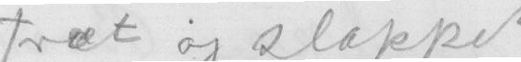Træt og slappet
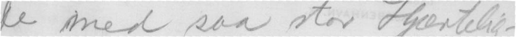de med saa stor Hjærtelig-
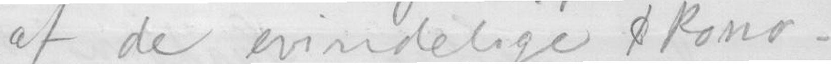af de evindelige & Pono-
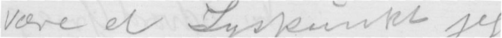være et Lyspunkt jeg
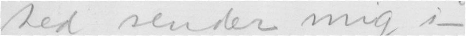hed sender mig i
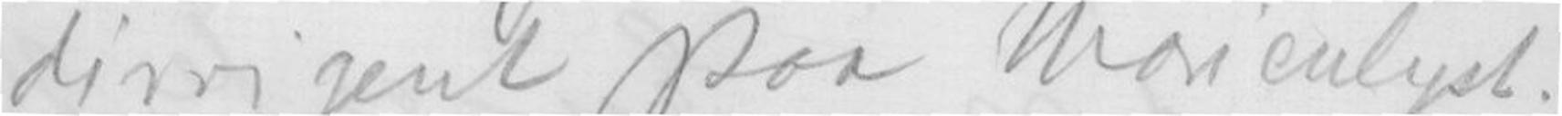dirrigent paa Marienlyst.
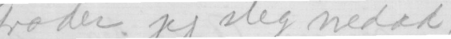grader jeg steg nedad,
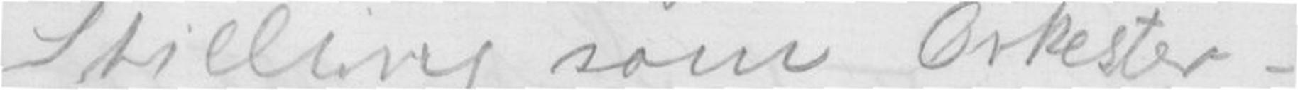Stilling som Orkester-
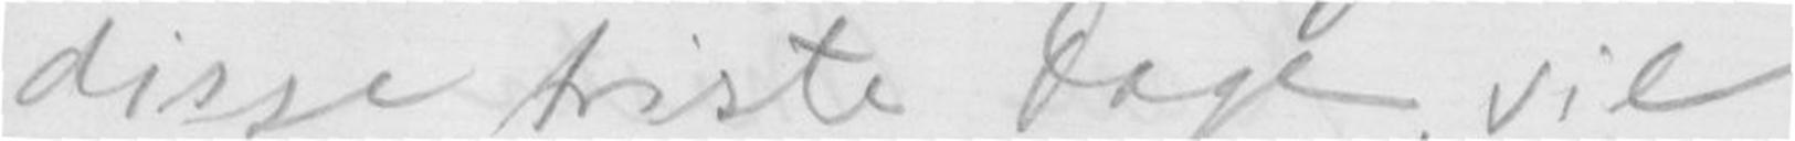disse triste dage, vil
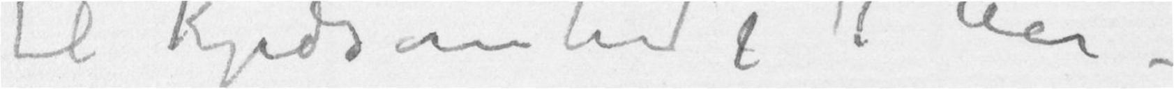til Kjedsomhed i de Aar –
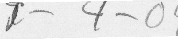54-4-09
